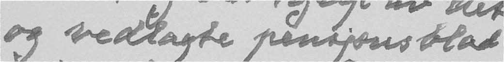og vedlagte pensjonsblad
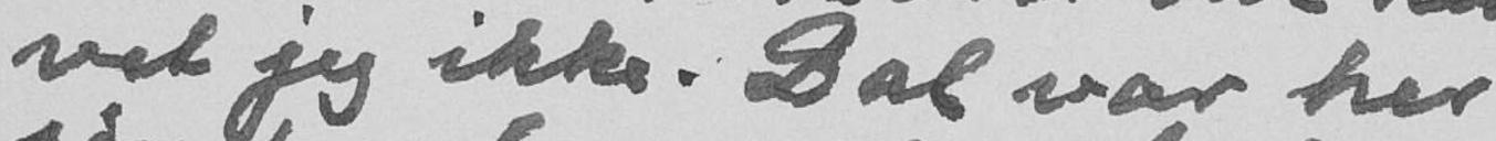vet jeg ikke. Dal var her
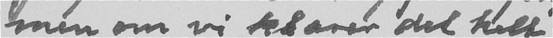men om vi klarer det helt
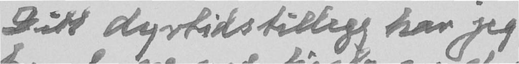Ditt dyrtidstillegg har jeg
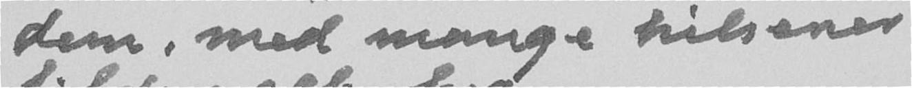dem, med mange hilsener
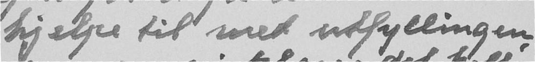hjelpe til med utfyllingen,
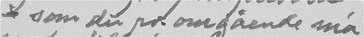- som du pr. omgående må
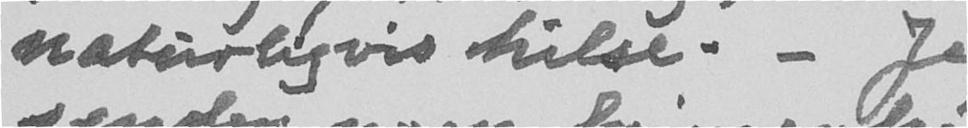naturligvis hilse. - Jeg
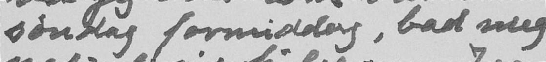søndag formiddag, bad meg
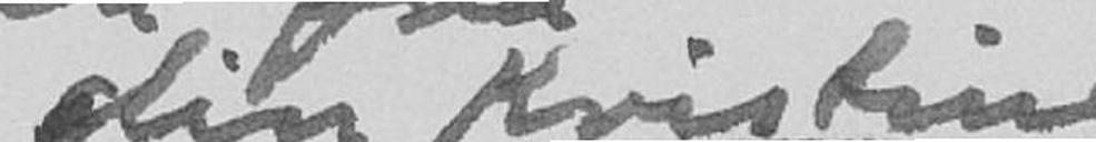din Kristine
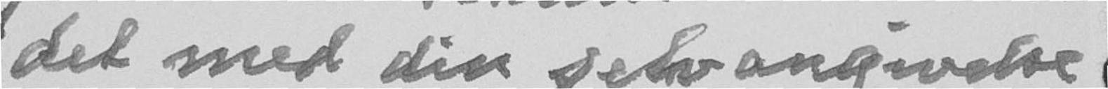det med din selvangivelse
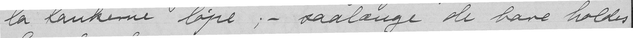la tankerne løpe ; - paalænge de bare holdes
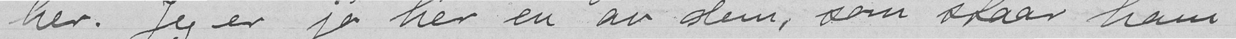her. Jeg er jo her en av dem, som staar ham
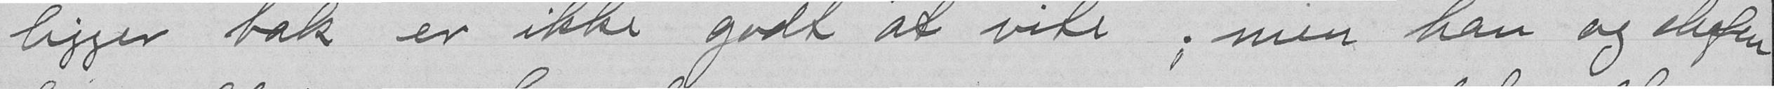ligger bak er ikke godt at vite ; men han og $
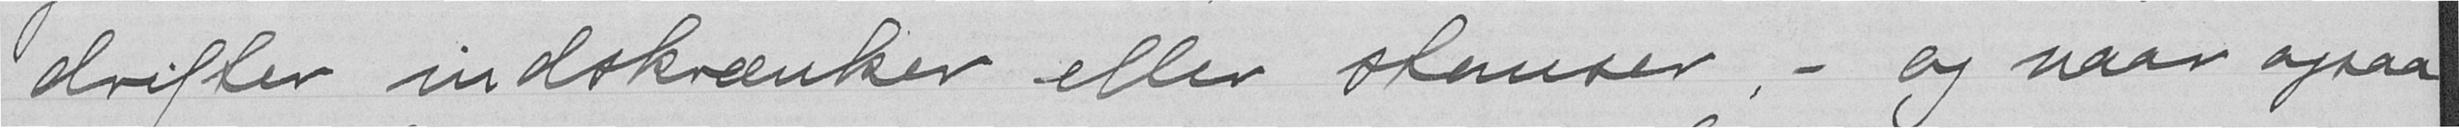drifter indskrænker eller slanser, - og naar ogsaa
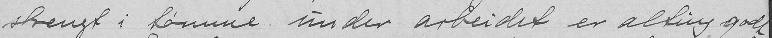strengt i tømme under arbeidet er alling godt
